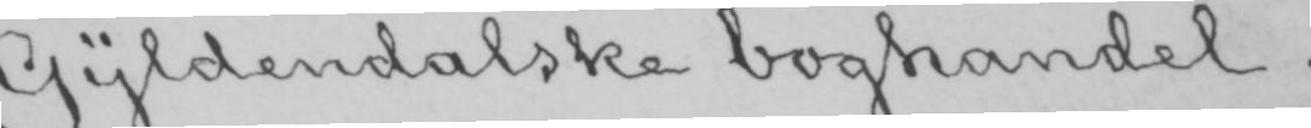Gyldendalske boghandel.
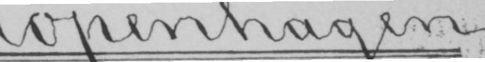Kopenhagen.
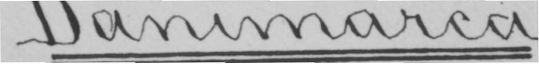(Danimarca.)
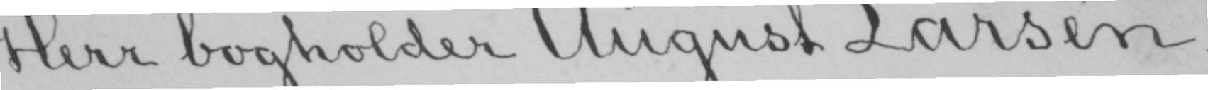Herr bogholder August Larsen.
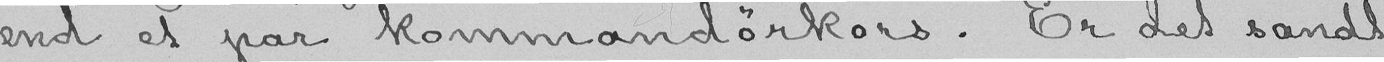end et par kommandørkors. Er det sandt
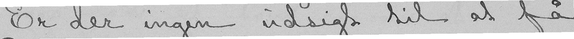Er der ingen udsigt til at få
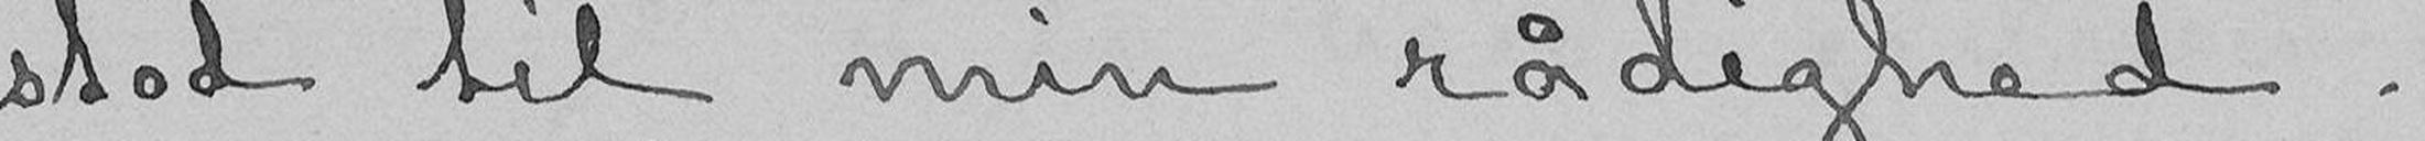stod til min rådighed.
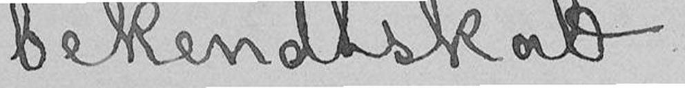bekendtskab.
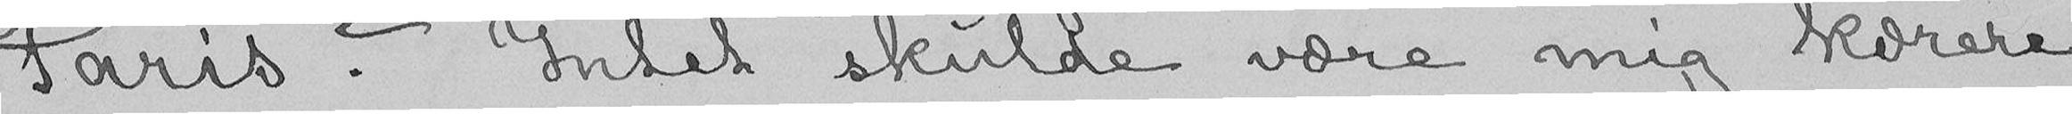Paris? Intet skulde være mig kærere
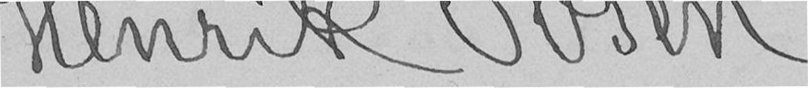Henrik Ibsen.
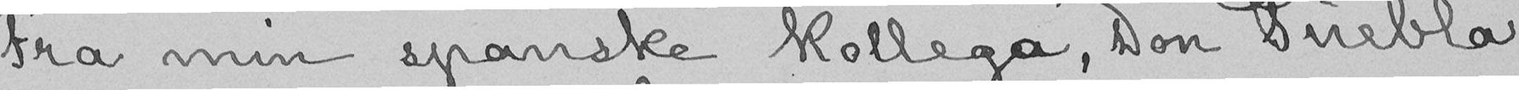Fra min spanske kollega, Don Puebla,
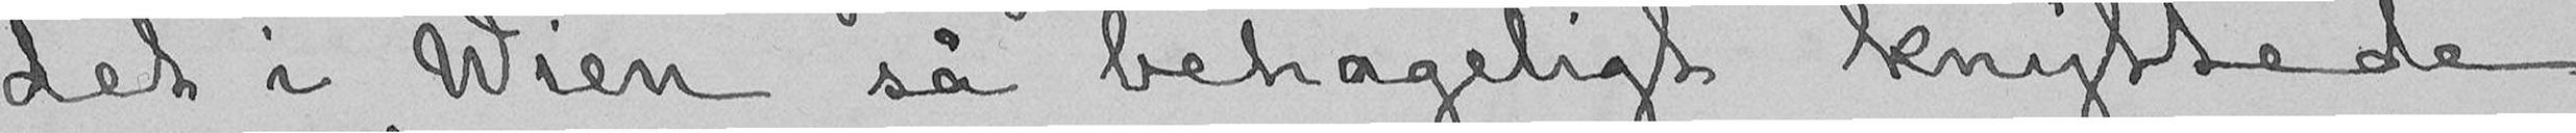det i Wien så behageligt knyttede
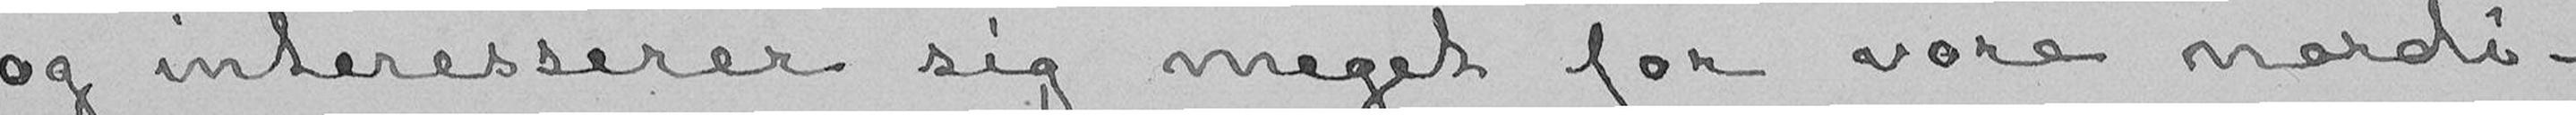og interesserer sig meget for vore nordi-
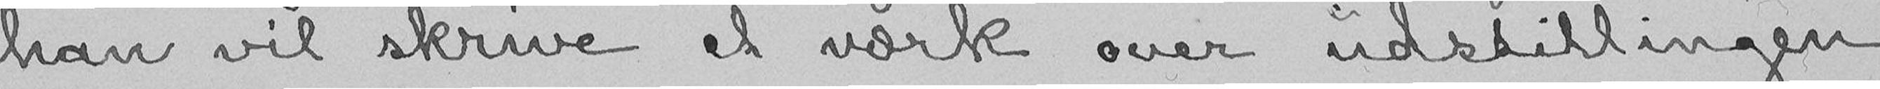han vil skrive et værk over udstillingen
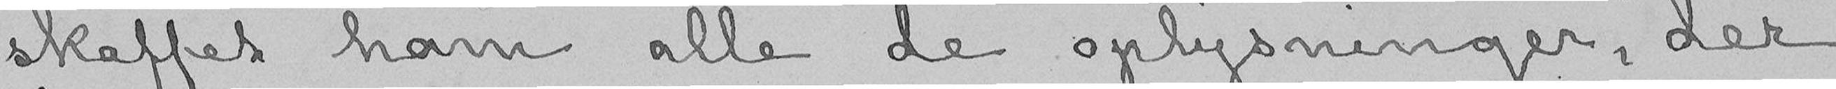skaffet ham alle de oplysninger, der
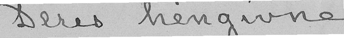Deres hengivne
